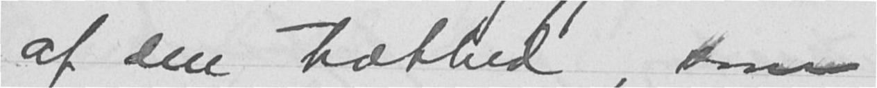af den træthed, som
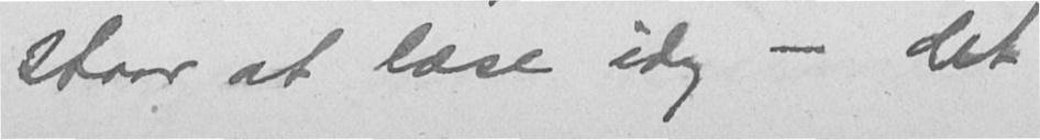staar at læse idag - det
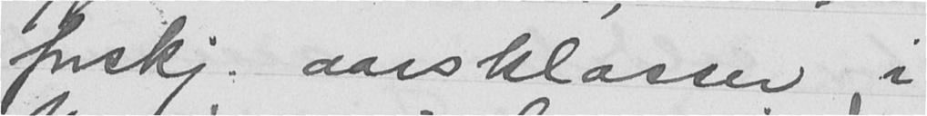forskj. aarsklasser i
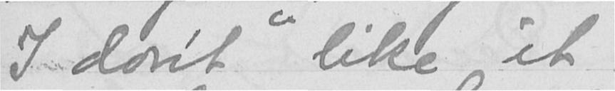I don't like it.
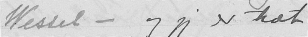Wessel - og jeg er træt
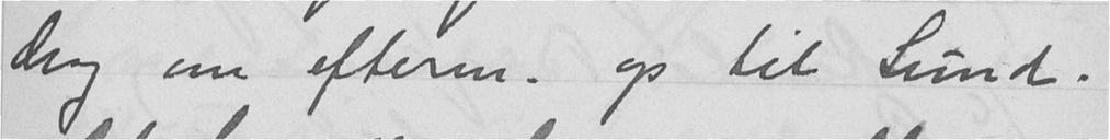drog om efterm. op til Sund.
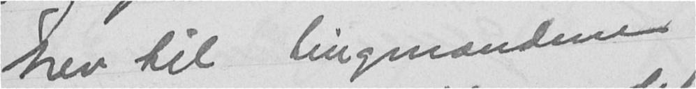brev til tingmænderne
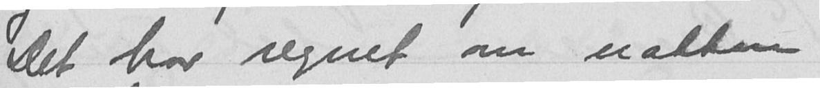Det har regnet om natten
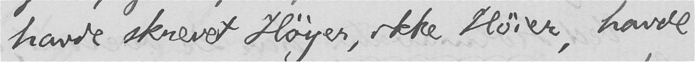havde skrevet Høyer, ikke Høier, havde
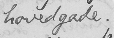hovedgade.
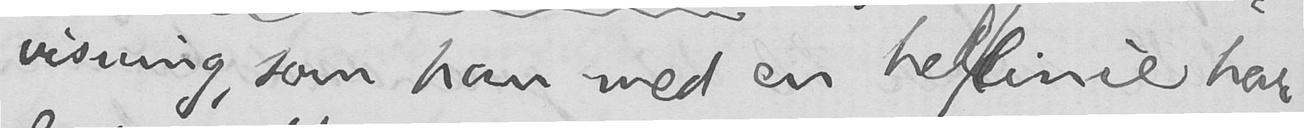visning, som han med en hel linie har
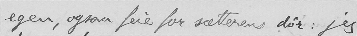egen, ogsaa feie for sætterens dør: jeg
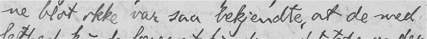ne blot ikke var saa bekjendte, at de med
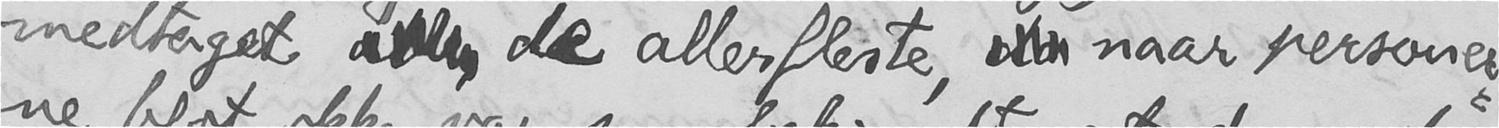medtaget  de aller fleste,  naar personer-
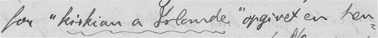for "kirkian a Islande" opgivet en hen-
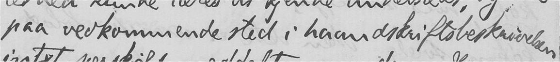paa vedkommende sted i haandskriftsbeskrivelsen
